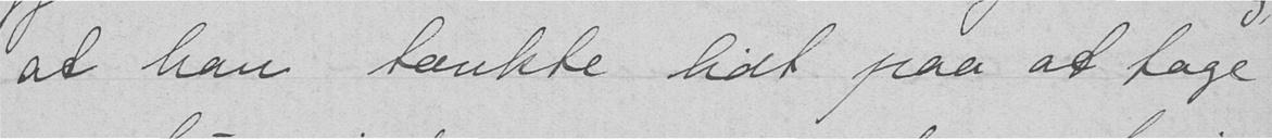at han tænkte lidt paa at tage
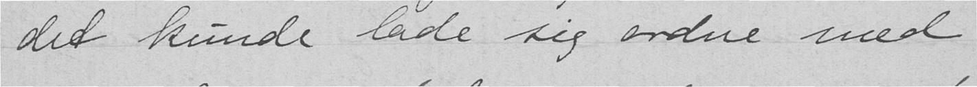det kunde lade sig ordne med
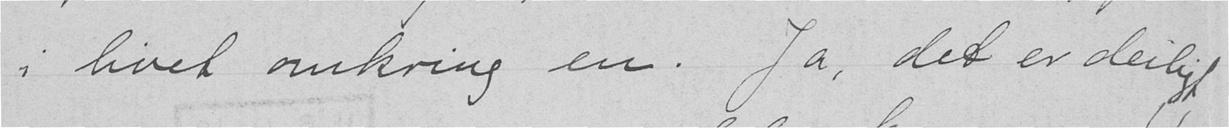i livet omkring en. Ja, det er deiligt
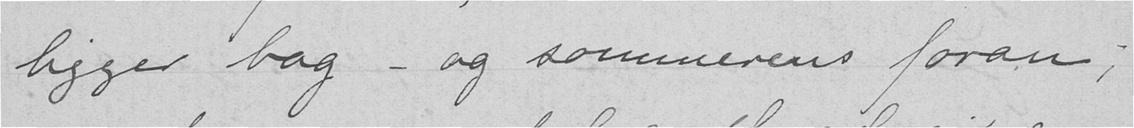ligger bag - og sommerens foran;
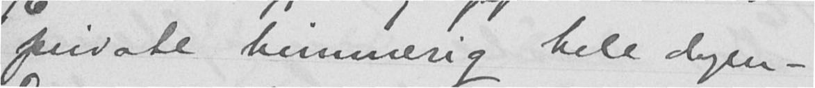private himmerig hele dagen -
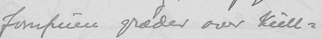Jomfruen græder over Kull-
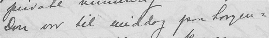Don var til middag paa Sorgen-
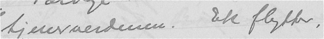tjenerverdenen. Ek flytter,
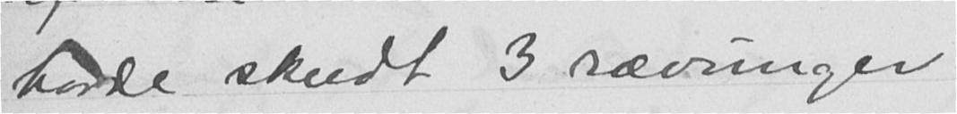havde skudt 3 rævunger
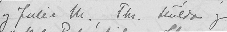og Julie M., Thr. Hulda og
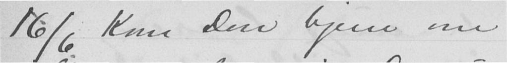16/6 Kom Don hjem om
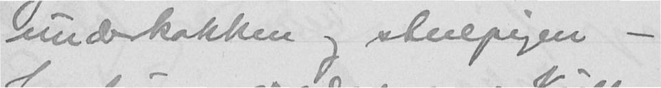underkokken og stuepigen -
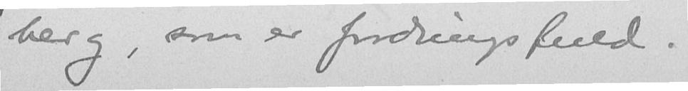berg, som er fordringsfuld.
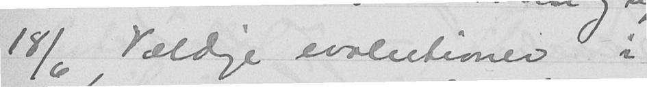18/6 Veldige evolutioner i
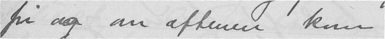fri og om aftenen kom
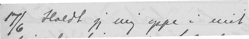17/6 Holdt jeg mig oppe i mit
